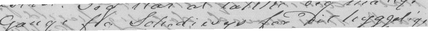Gange fra Schediwys for Dit hyggelige
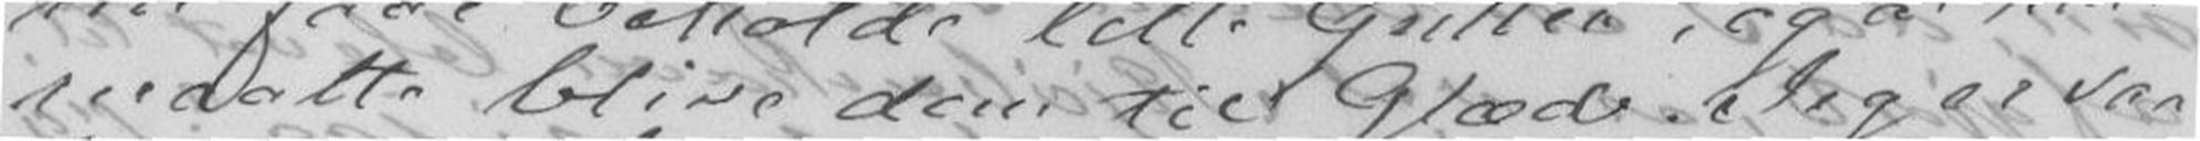maatte blive til Glæde. Jeg er saa
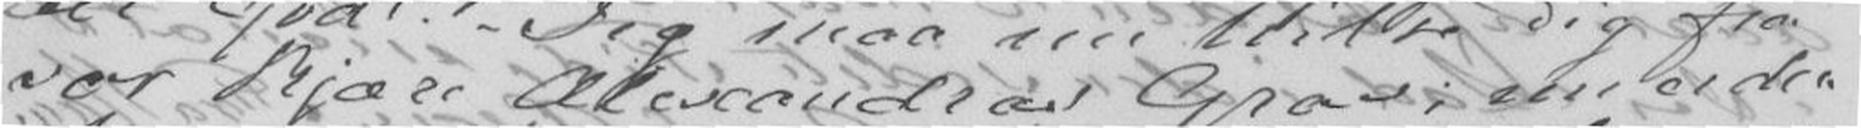vor kjære Alexandras Grav; nu er den
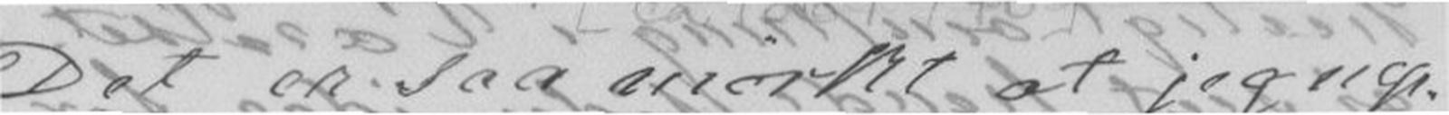Det er saa mørkt at jeg nep-
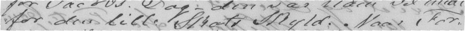for den lille Skats Skyld. Naar For-
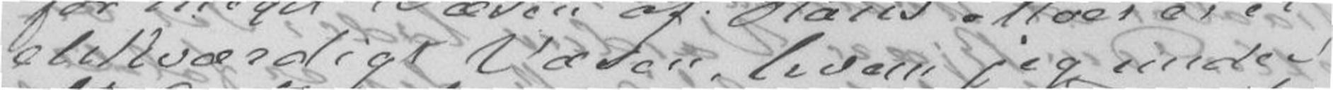elskværdigt Væsen, hvem jeg under
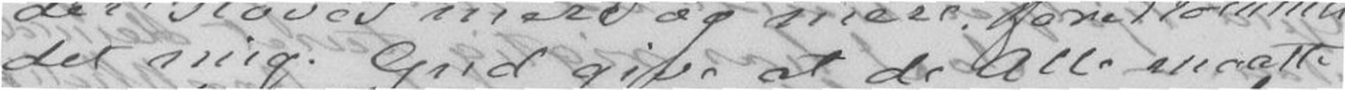det mig. Gud give at de Alle maatte
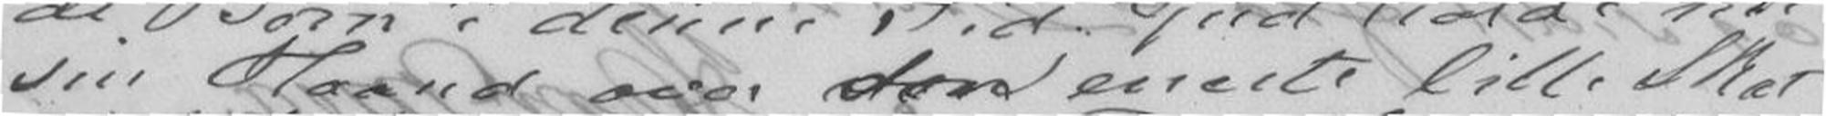sin haand over den eneste lille Skat
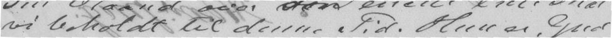vi beholdt til denne Tid. Hun er, Gud
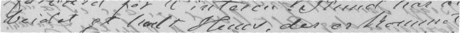beidet et heelt Huus, der er kommet
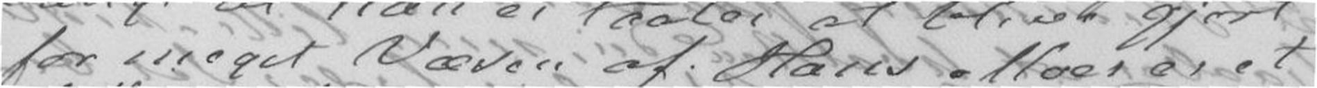for meget Væsen af Hans Moer er et
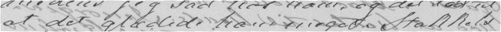at glædedeham meget, Stakkels
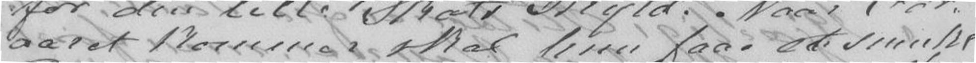aaret kommer skal hun faae et snukt
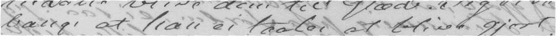bange at han ei taaler at blive gjort
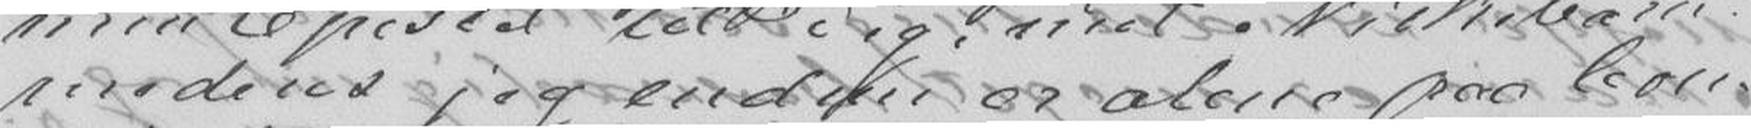medens jeg endnu er alene paa Con-
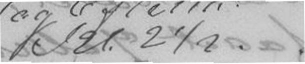Kl.2½.
